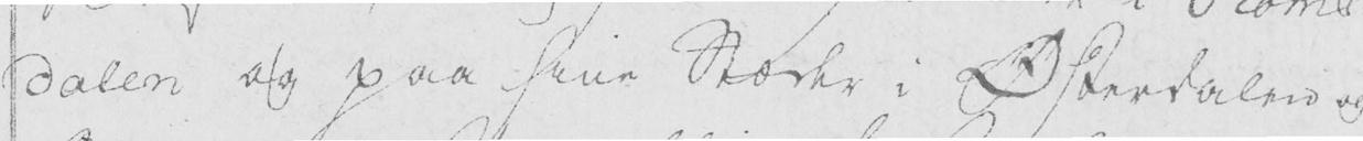dalen og paa hine Stæder i Østerdalen og
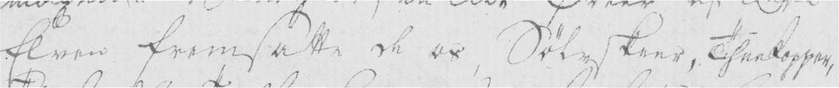Elven fremsatte de os, Sølvskeer, Theekopper,
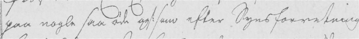paa nogle saa øde og som efter Synsforretning
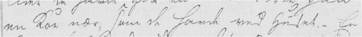en Kor nær, som de havde ved Huset. - En
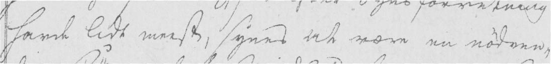havde lidt meest, synes at være en nødven-
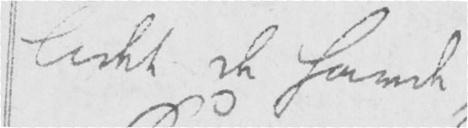lidet de havde
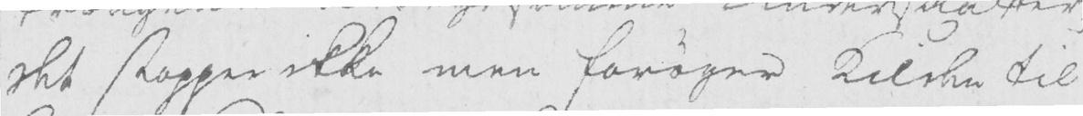det stopper ikke men forøger Kilden til
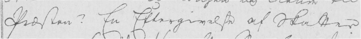Præsten? En Eftergivelse af Skatter
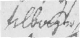tilbage
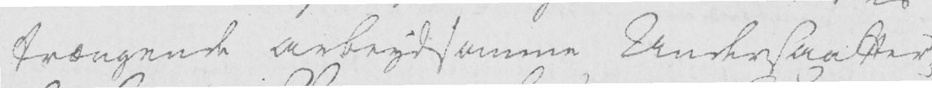trængende arbeydsomme Undersaatter;
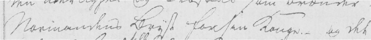Normandens Bryst for sin Konge. - og det
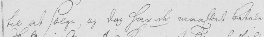til at sælge, og dog har de maattet betale
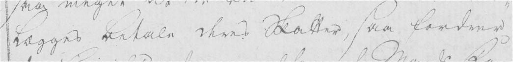lægges betale deres Skatter, saa fordrer
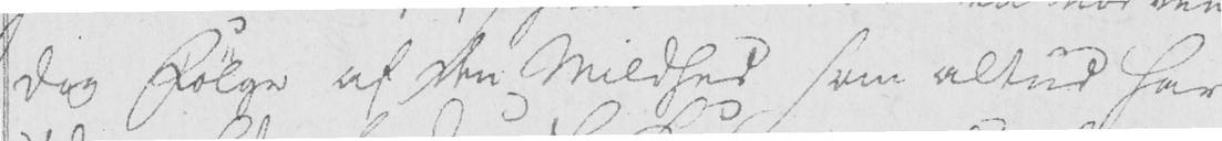dig Følge af den Mildhed som altid har
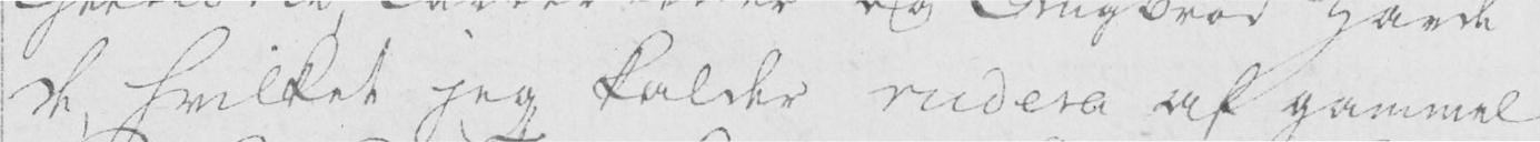de, hvilket jeg kalder rudera af gammel
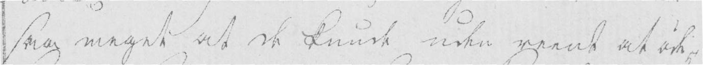saa meget at de kunde uden reent at øde-
